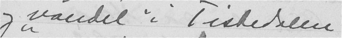og "vandel" i Tistedalen
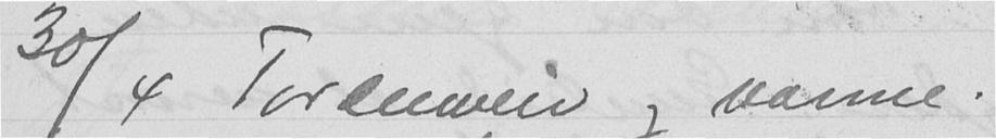30/4 Tordenveir og varme.
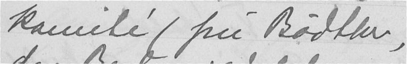komité (fru Bødtker,
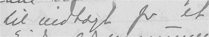til indtægt for et
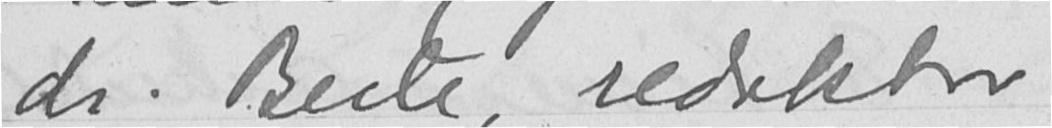dr. Berle, redaktør
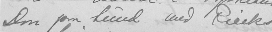Don paa Sund med Riecks
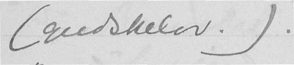(gudskelov.).
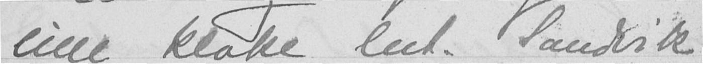lille kloke lnt. Sandvik
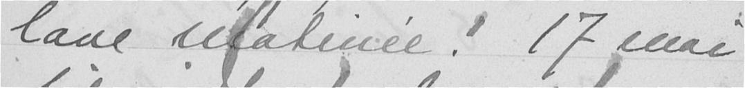lave matinée! 17 mai
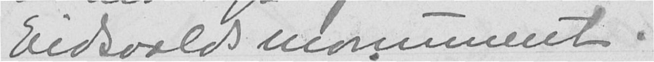Eidsvoldsmonument.
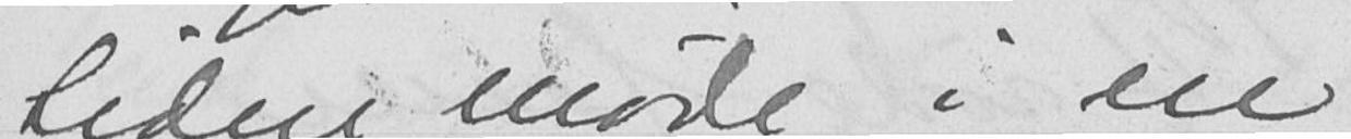Siden møde i en
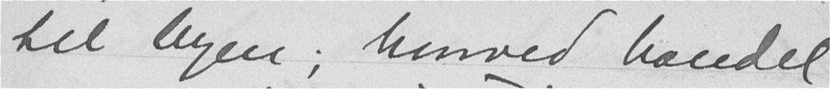til byen; hvorved handel
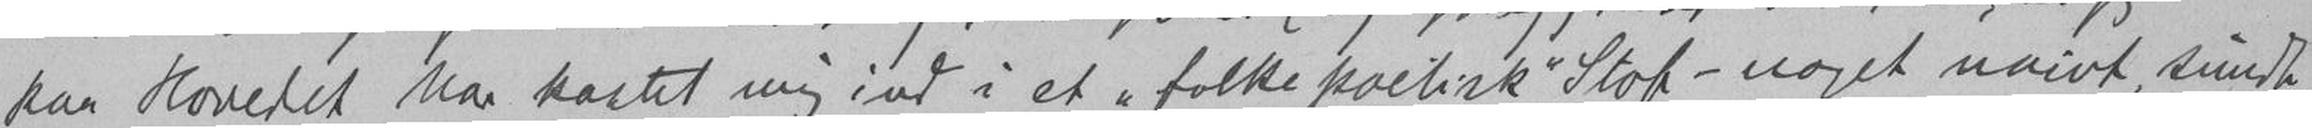paa Hovedet har kastet mig ind i et "folkepoetisk" Stof - noget naivt, sundt
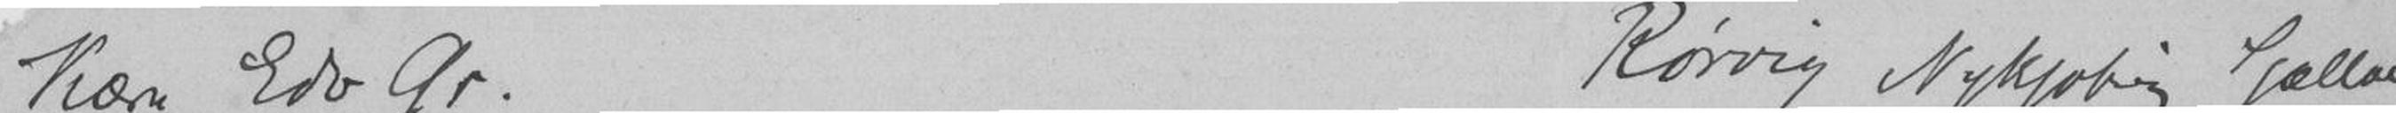Kære Edv Gr. Rørvig Nykjøbing Sjælland
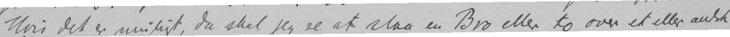Hvis det er muligt, da skal jeg se at slaa en Bro eller to over et eller andet
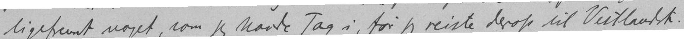ligefremt noget, som jeg havde Tag i, før jeg reiste derop til Vestlandet.
